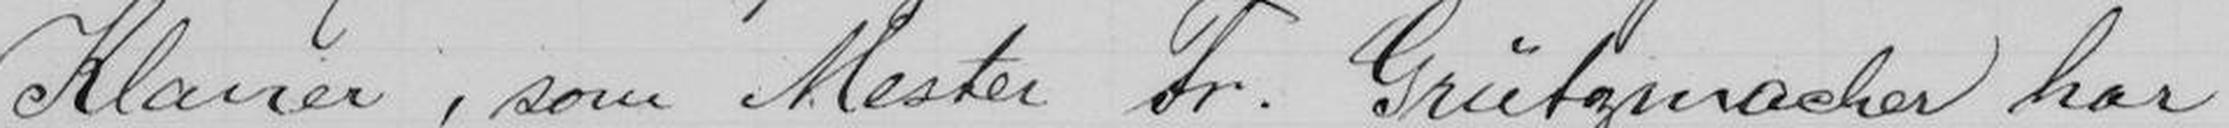Klaver, som Mester Fr. Grützmacher har
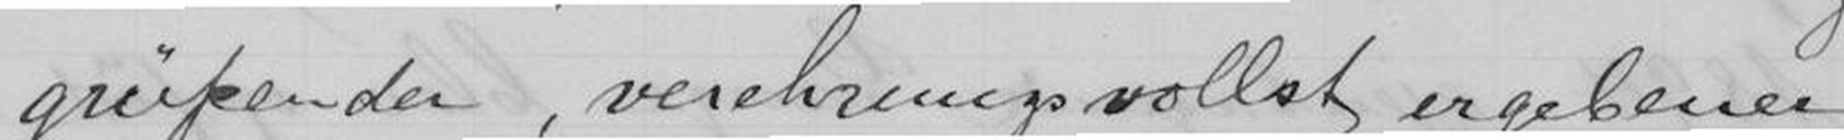grüssender, verchrungsvollst ergebener
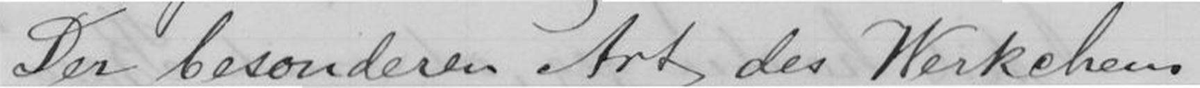Der besonderen Art des Werkchens
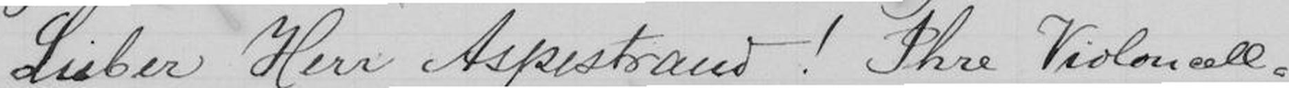"Lieber Herr Aspestrand! Ihre Violoncell-
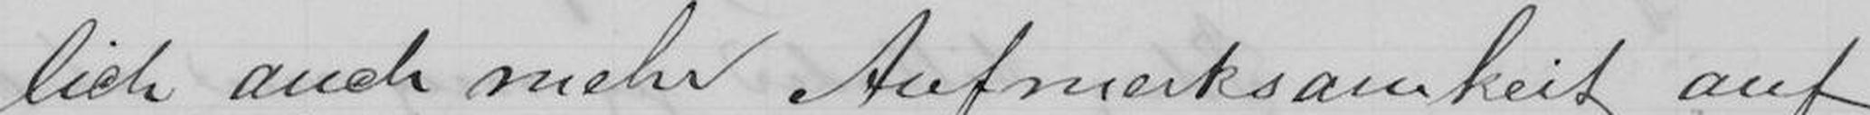lich auch mehr Aufmerksamkeit auf
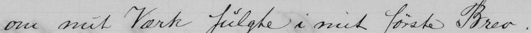om mit Værk fulgte i mit første Brev.
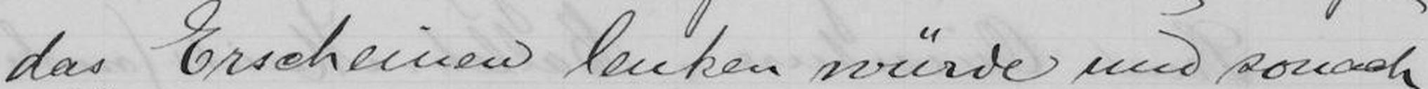das Erscheinen lenken mürde und sonach
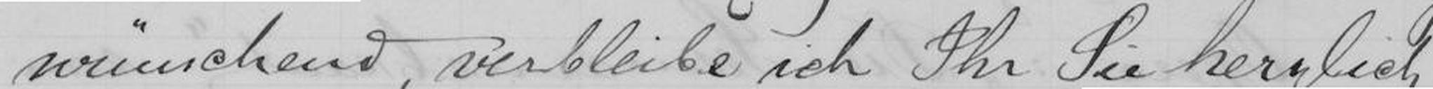wümchend, verbleibe ich Ihr Sie herzlich
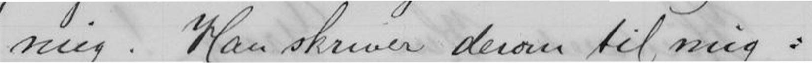mig. Han skriver derom til mig:
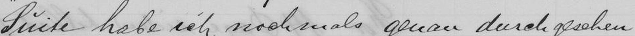Suite habe ich nach mals genan durchgeschen
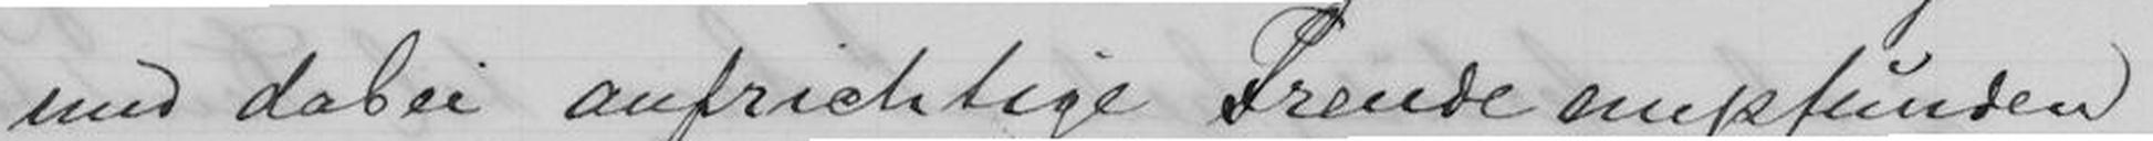und dabei aufrichtige Frende empfunden
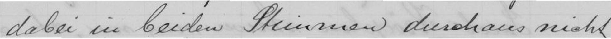dabei in beiden Stimmen durchaus nicht
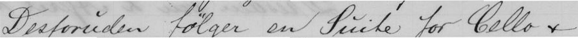Desforuden følger en Suite for Cello &
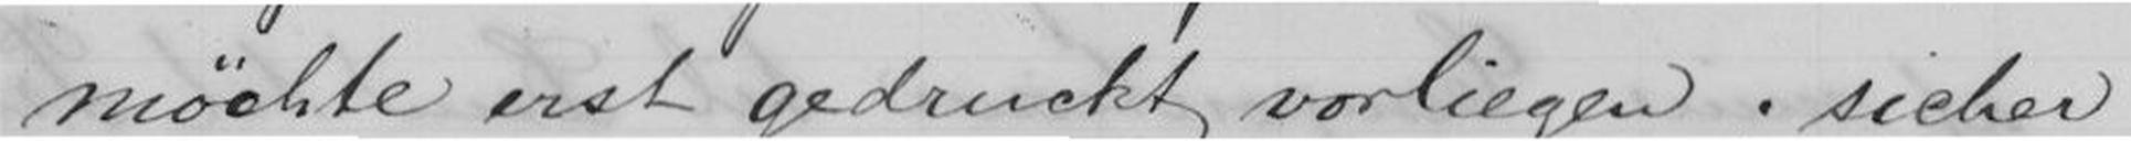möchte erst gedrucht vorliegen; sicher
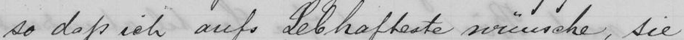so daß ich aufs Lebhafteste wünsche, sie
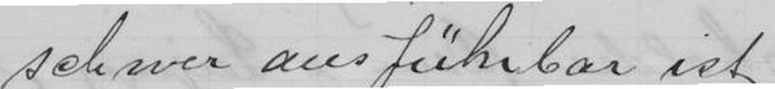schwer ausführbar ist.
